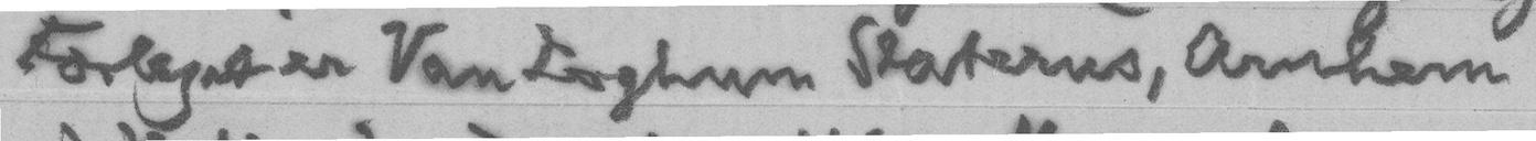Forlaget er Van Loghum Slaterus, Arnhem
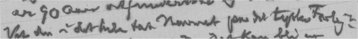Vet du i det hele tat Navnet paa det tyske Forlag?
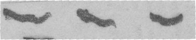- - -
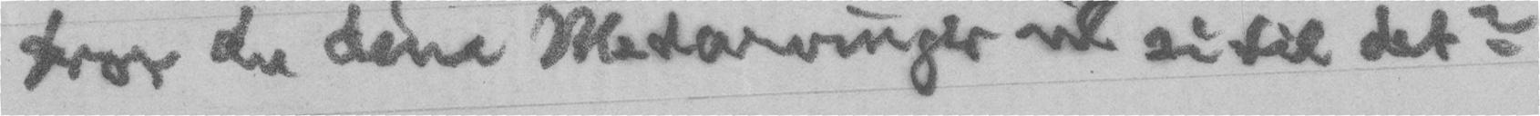tror du dine Medarvinger vil si til det?
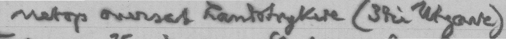netop oversat Landstrykere (3die Utgave)
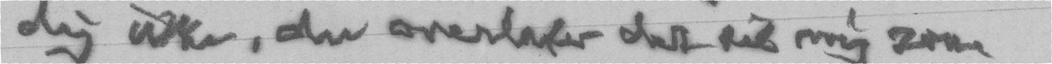dig ikke, du overlater det til mig som
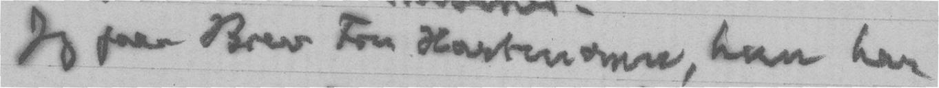Jeg faar Brev Fru Hartensmre, hun har
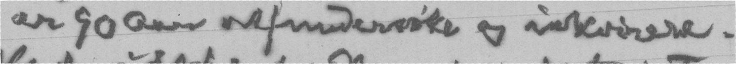er 90 Aar at undersøke og inkassere.
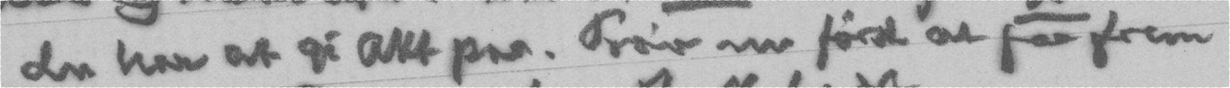du har at gi Akt paa. Prøv nu først at faa frem
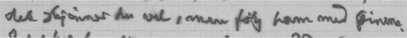det skjønner du vel, men følg ham med Øinene.
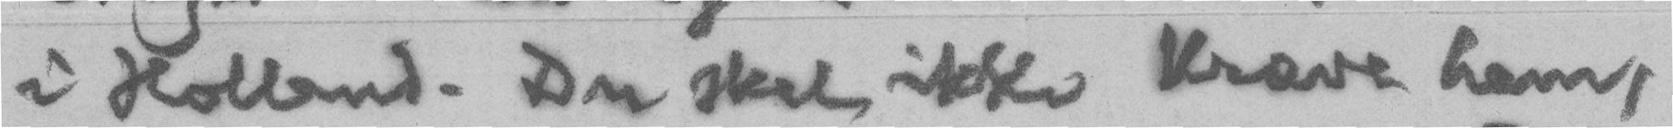i Holland. Du skal ikke kræve ham,
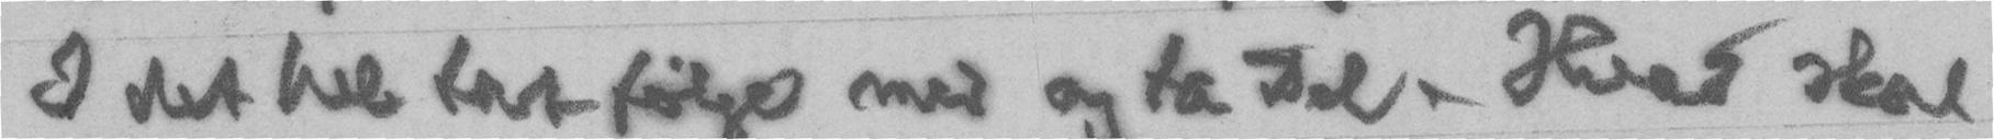I det hel tat følge med og ta Del. Hvad skal
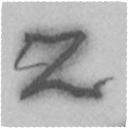2
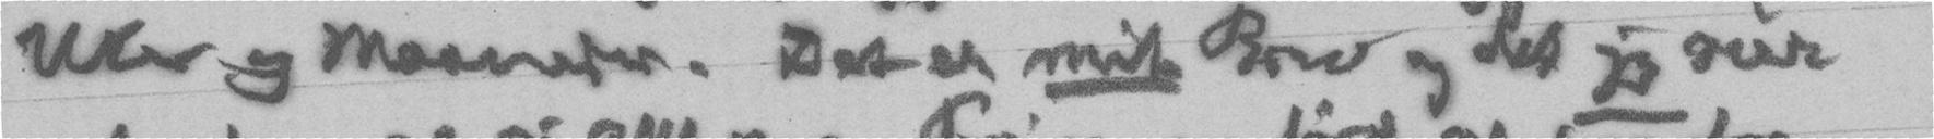Uker og Maaneder. Det er mit Brev og det jeg sier
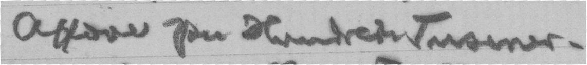Affære paa HundredeTusener.
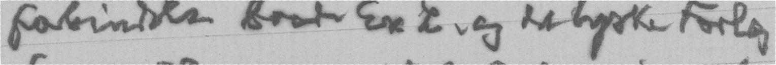forbindelse baade Ex L. og det tyske Forlag
